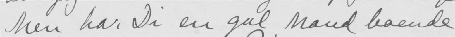Men har Di en gal Mand boende
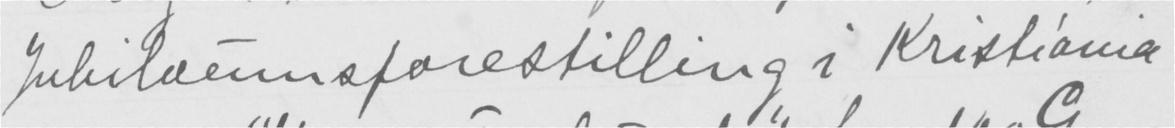Jubilæumsforestilling i Kristiania
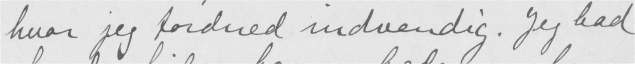hvor jeg tordned indvendig. Jeg bad
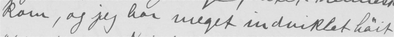kom, og jeg bor meget indviklet høit
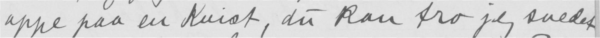oppe paa en Kvist, du kan tro jeg svedet
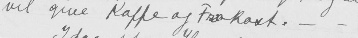vil give Kaffe og Frokost. — —
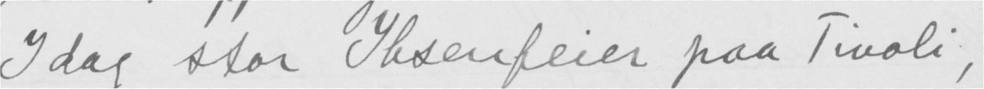Idag stor Ibsenseier paa Tivoli,
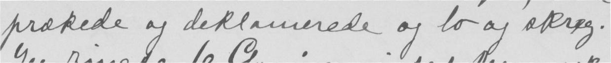prækede og deklamerede og lo og skreg.
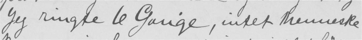Jeg ringte 6 Gange, intet Menneske
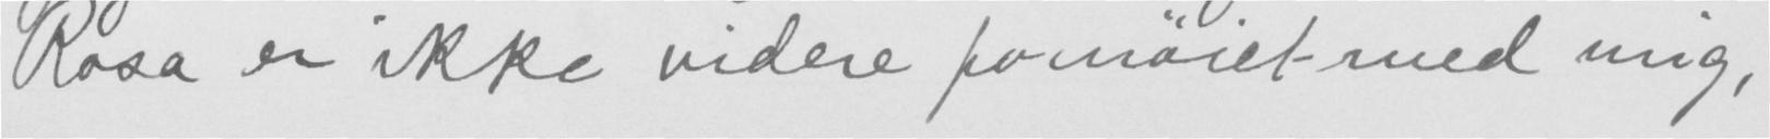Rosa er ikke videre fornøiet med mig,
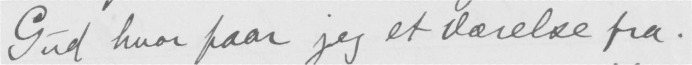Gud hvor faar jeg et Værelse fra.
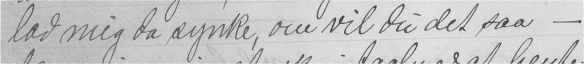lad mig da synke, om vil du det saa -
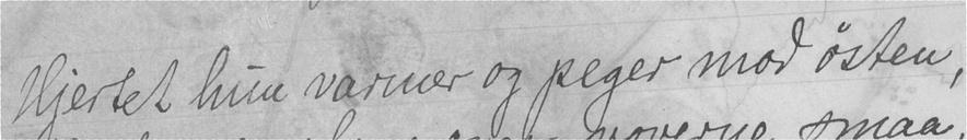Hjertet hun varmer og peger mod østen,
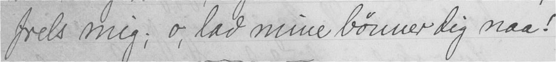frels mig; o, lad mine bønner dig naa!
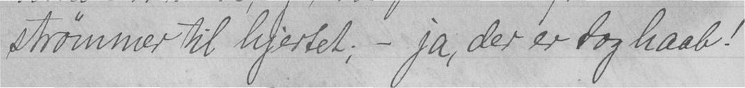strømmer til hjertet; - ja, der er dog haab!
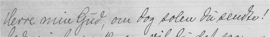Herre min Gud, om dog solen du sendte!
